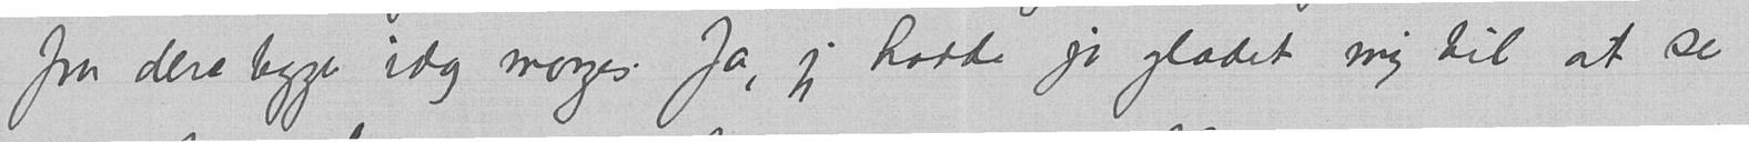fra dere begge idag morges. Ja, jeg hadde jo glædet mig til at se
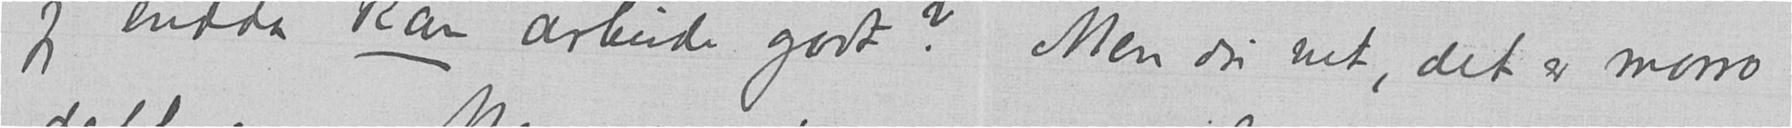jeg endda kan arbeide godt? Men du vet, det er morro
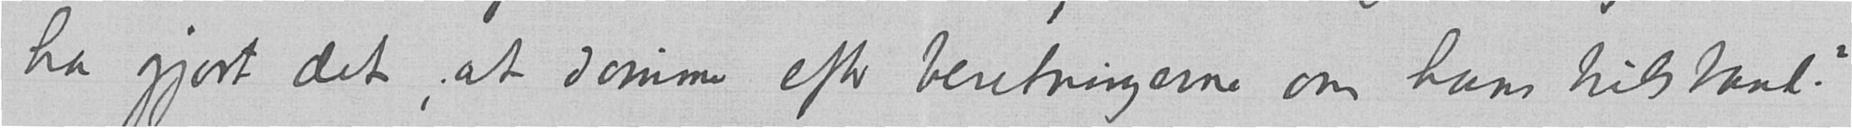ha gjort det, at dømme efter beretningerne om hans tilstand?
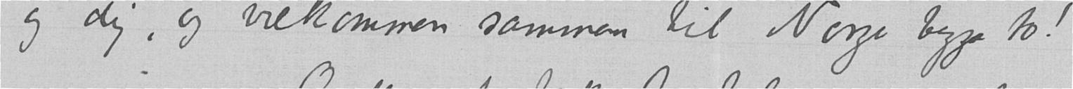og dig, og velkommen sammen til Norge begge to!
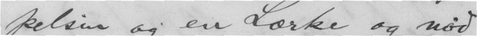pelsin og en Lærke og nød
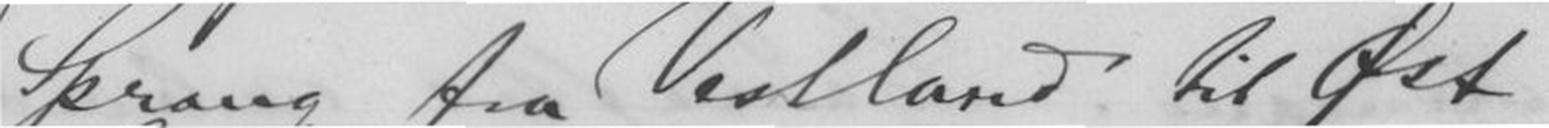Sprang fra Vestland til Øst-
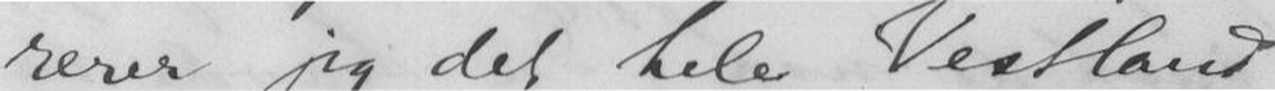rerer jeg det hele Vestland
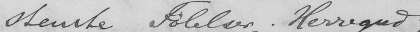stemte Følelser. Herregud,
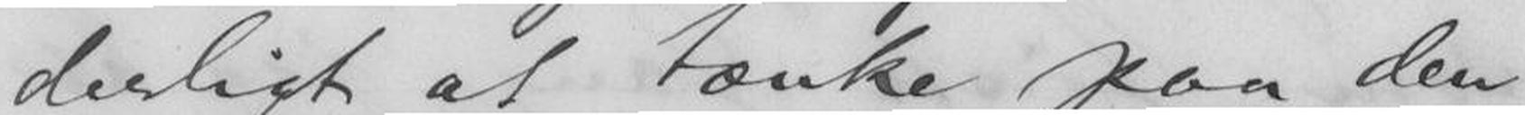derligt at tænke paa den
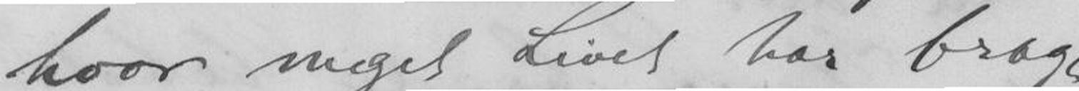hvor meget Livet har bragt
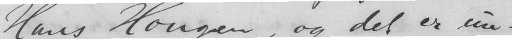Hans Hougen, og det er un
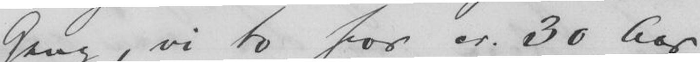Gang, vi to for ca. 30 Aar
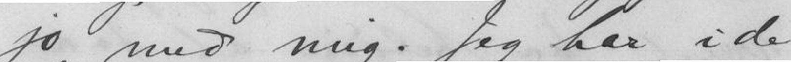jo med mig. Jeg har i de
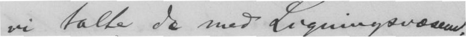vi talte da med Ligningsvæsenet
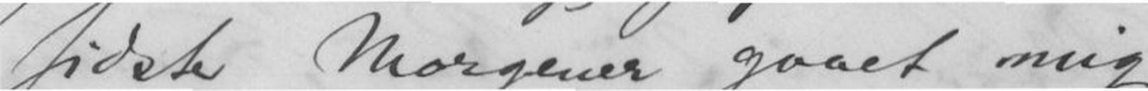sidste Morgener gaaet mig
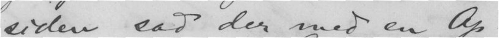siden sad der med en Ap-
